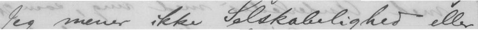Jeg mener ikke Selskabelighed eller
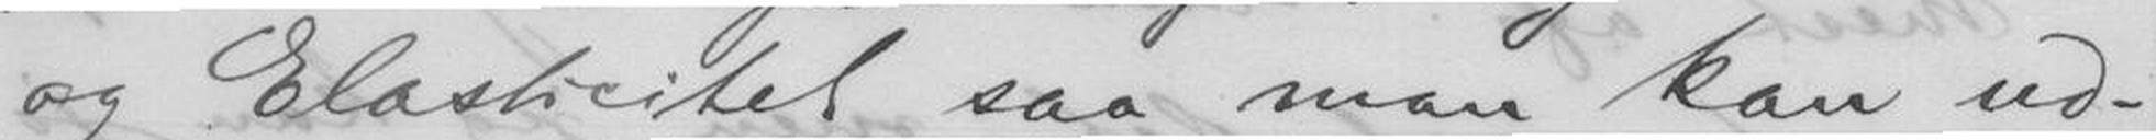og Elasticitet saa man kan ud-
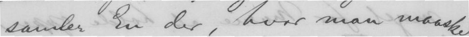samler En der, hvor man maaske
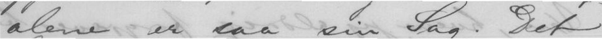alene er saa sin Sag. Det
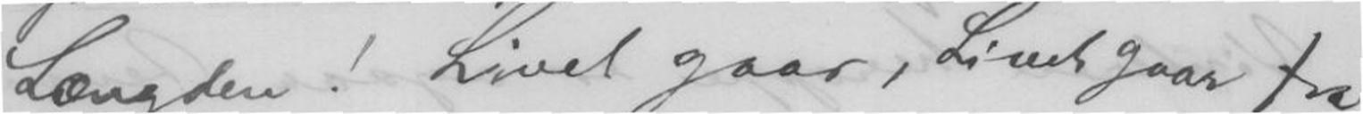Længden! Livet gaar, Livet gaar fra
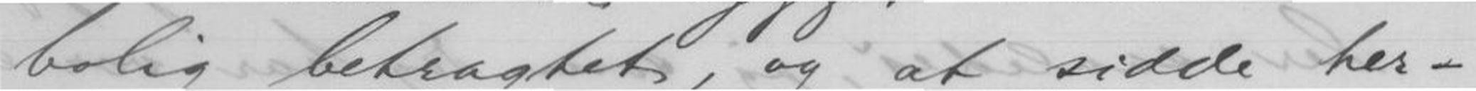bolig betragtet, og at sidde her-
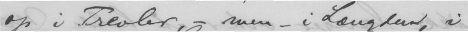op i Trevler, - men - i Længden, i
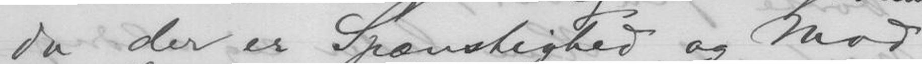da der er Spænstighed og Mod
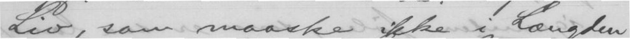Liv, som maaske ikke i Længden
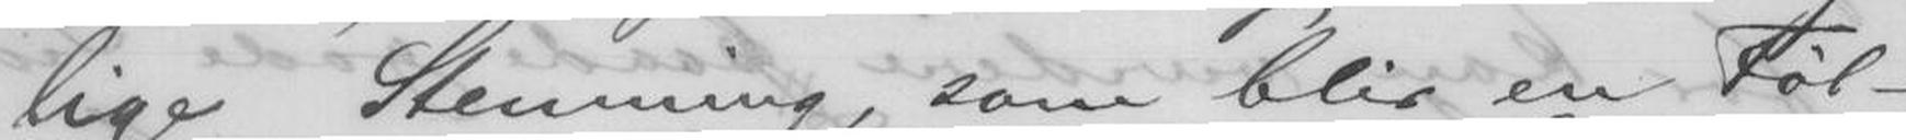lige Stemning, som blir en Føl-
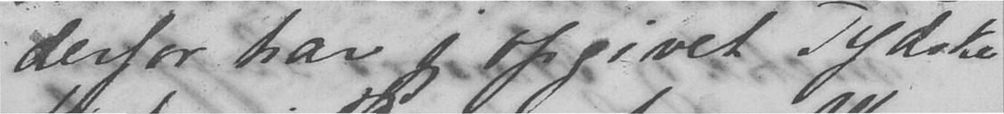derfor har jeg opgivet Tydske-
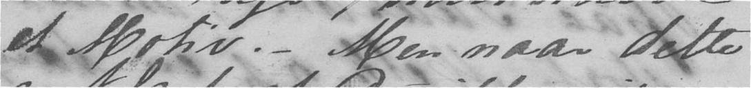et Motiv. - Men naar dette
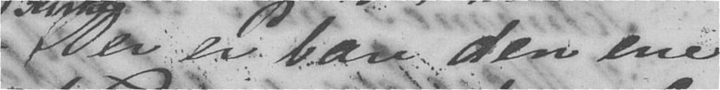Der er bare den ene
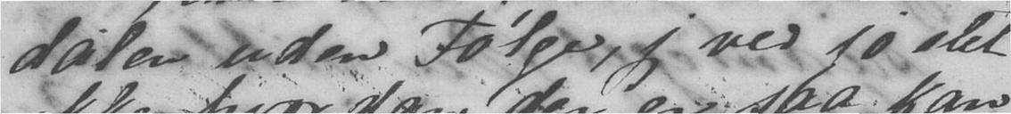dalen uden Følge, jeg ved jo slet
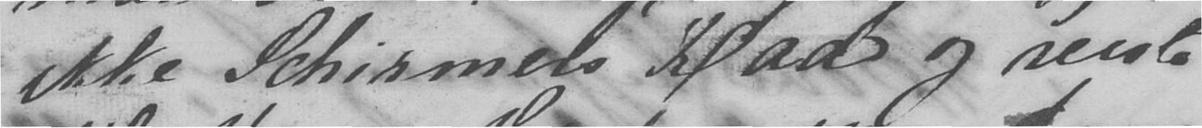ikke Schirmers Raad og reiste
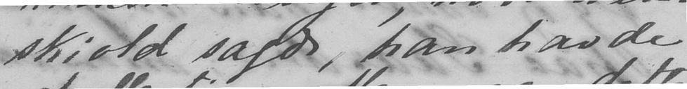skiold sagde, han havde
